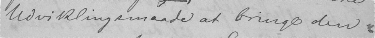Udviklingsmaade at bringe den
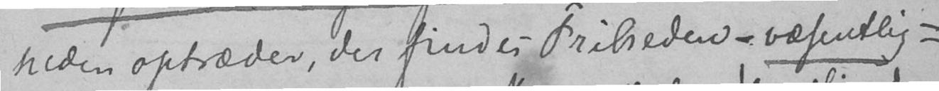heden optræder, der findes Friheden – væsentlig =
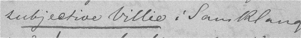subjective Villie i Samklang
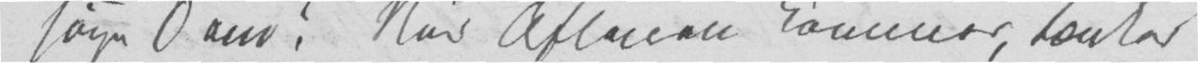søge Dem! Når Aftenen kommer, tænker
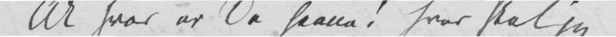Ak hvor er De henne! Hvor skal jeg
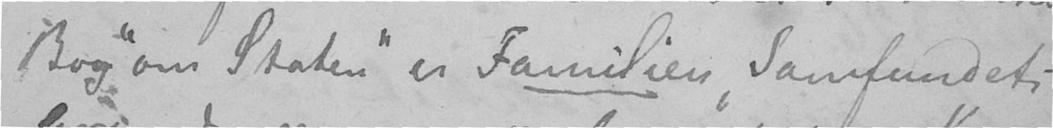Bog om Staten er Familien Samfundets
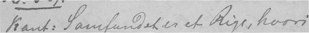Kant: Samfundet er et Rige, hvori
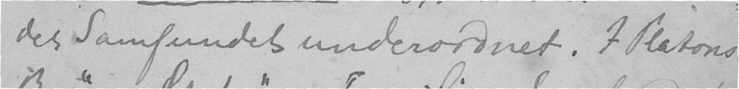det Samfundet underordnet. I Platons
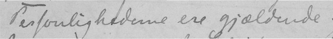Personlighederne ere gjældende.
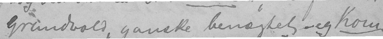Grundvold, ganske benægtet og Kom-
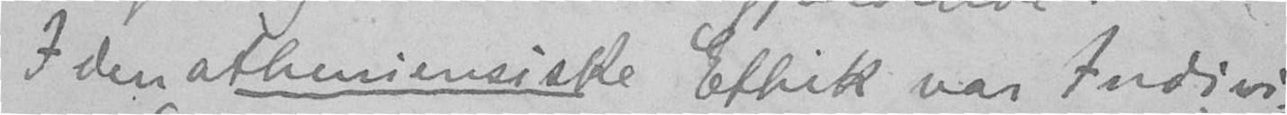I den atheniensiske Ethik var Indivi-
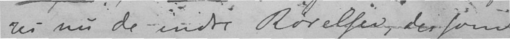res nu de indre Rørelser, dersom
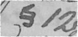§ 12
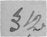§ 12
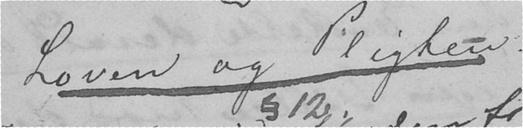Loven og Pligten
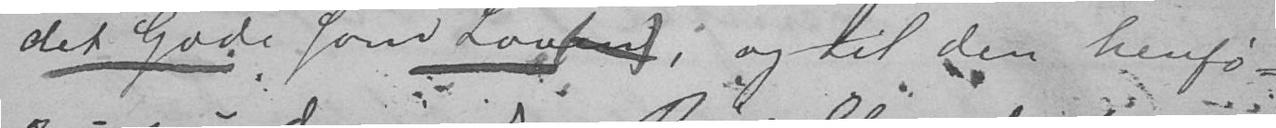det Gode som Loven, og til den henfø-
